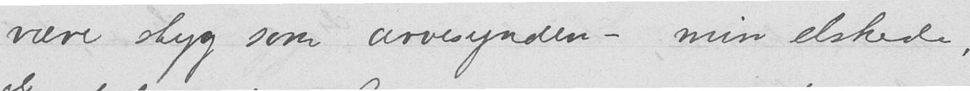være styg som arvesynden - min elskede,
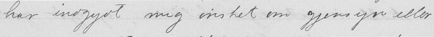har indgydt mig ønsket om gjensyn eller
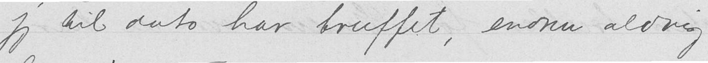jeg til dato har truffet, endnu aldrig
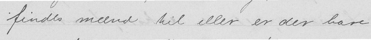findes mænd til eller er der bare
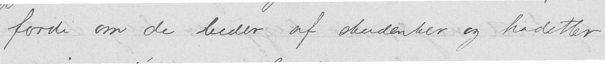fordi om de beder af studenter og kadetter
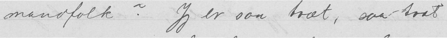mandfolk? Jeg er saa træt, saa træt
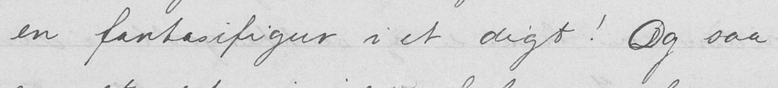en fantasifigur i et digt! Og saa
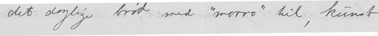det daglige brød med "morro" til, kunst
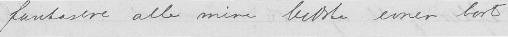fantasere alle mine bedste evner bort
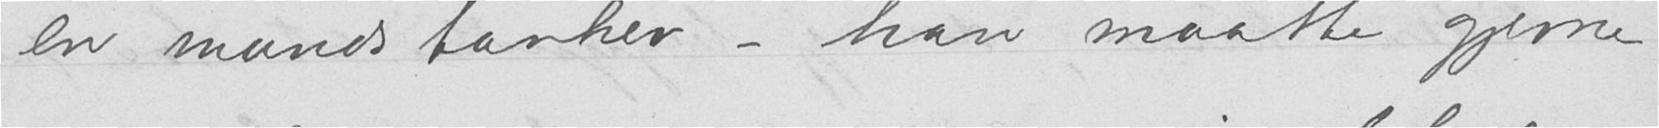en mands tanker - han maatte gjerne
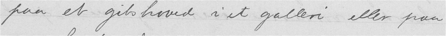paa et gibshoved i et galleri eller paa
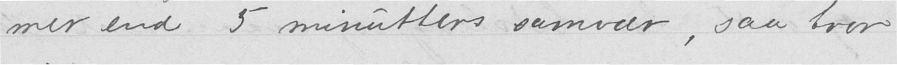mer end 5 minutters samvær, saa tror
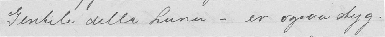Gentile della Luna - er også styg.
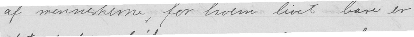af menneskerne, for hvem livet bare er
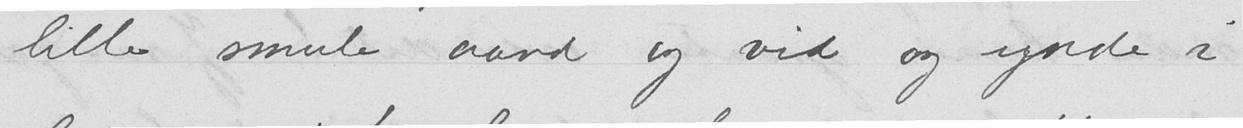lille smule aand og vid og ynde i
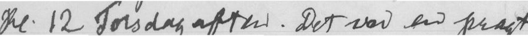Kl. 12 Torsdag aften. Det var en pragt-
